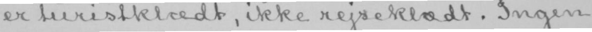er turistklædt, ikke rejseklædt. Ingen
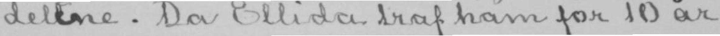dellene. Da Ellida traf ham for 10 år
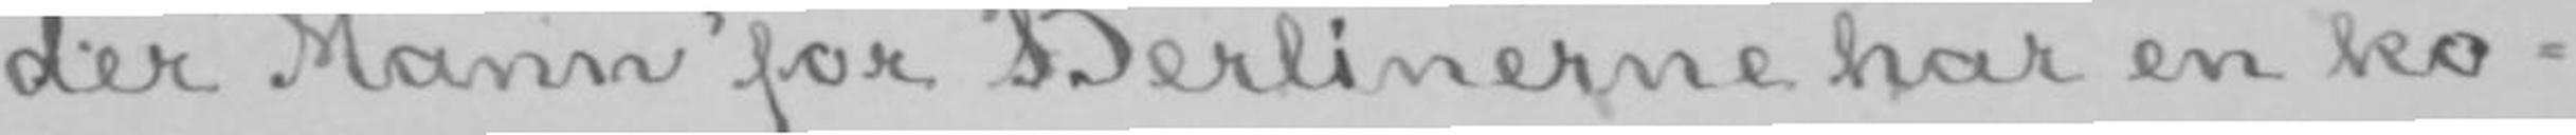der Mann» for Berlinerne har en ko-
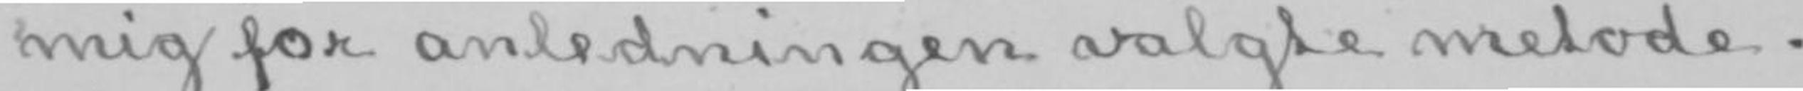mig for anledningen valgte metode.
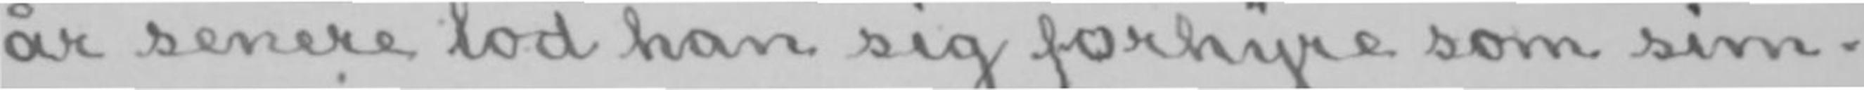år senere lod han sig forhyre som sim-
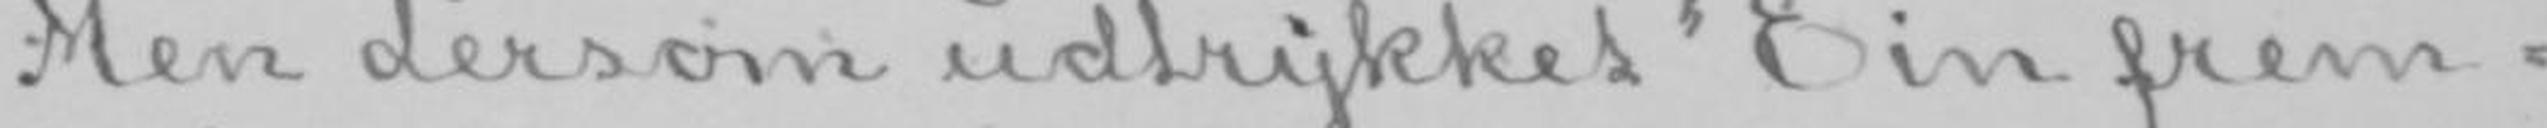Men dersom udtrykket «Ein frem-
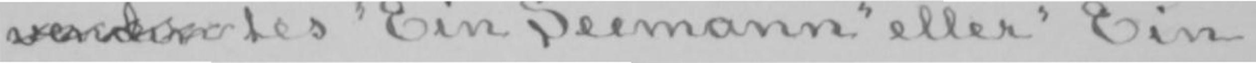vendintes «Ein Seemann» eller «Ein
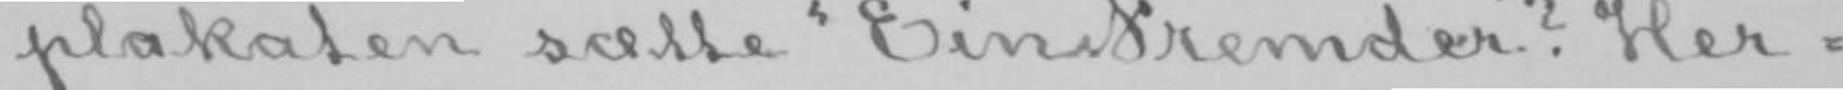plakaten sætte «Ein Fremder»? Her-
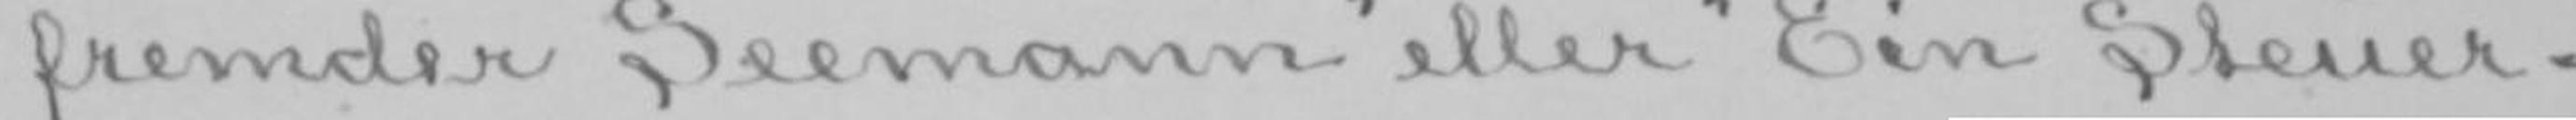fremder Seemann» eller «Ein Steuer-
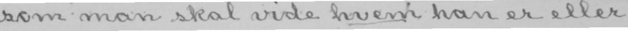som man skal vide hvem han er eller
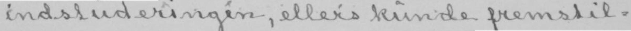indstuderingen, ellers kunde fremstil-
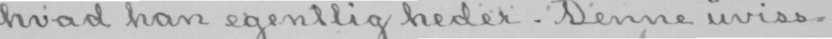hvad han egentlig heder. Denne uviss-
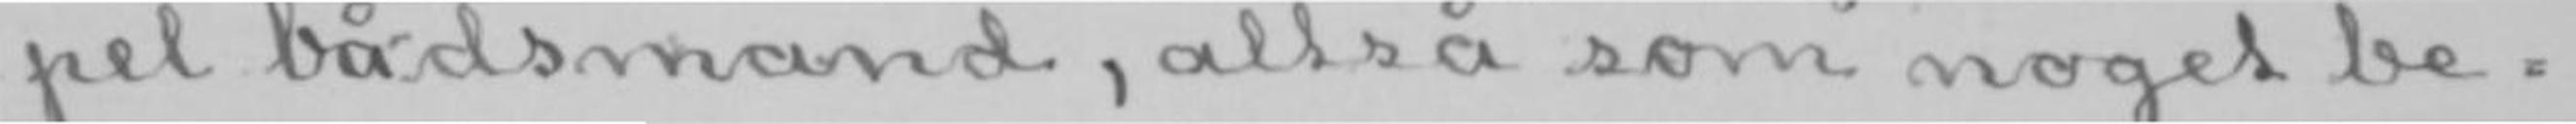pel bådsmand, altså som noget be-
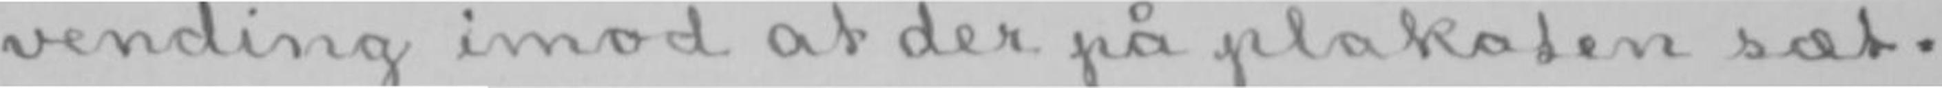vending imod at der på plakaten sæt-
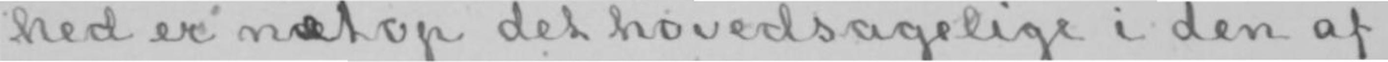hed er netop det hovedsagelige i den af
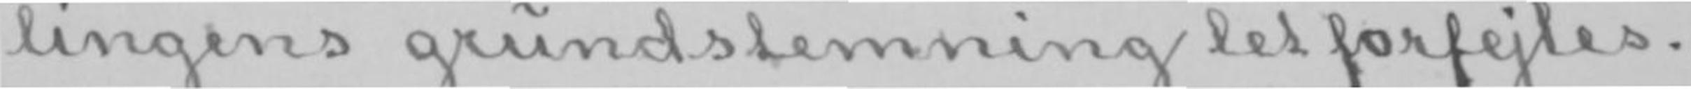lingens grundstemning let forfejles.
